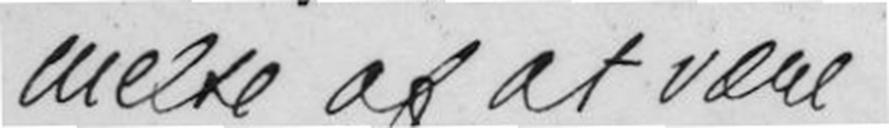melse af at være
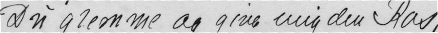Du glemme og give mig den Ros,
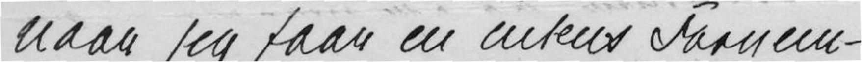naar jeg faar en intens Foruan-
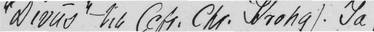"Divus" til C Chr. Krohg. Ja,
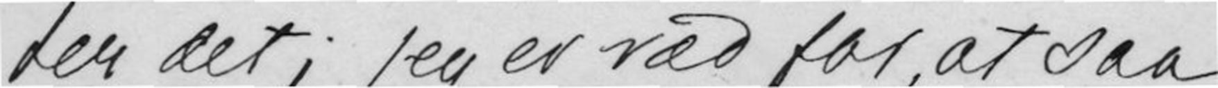der det; jeg er ræd for, at saa
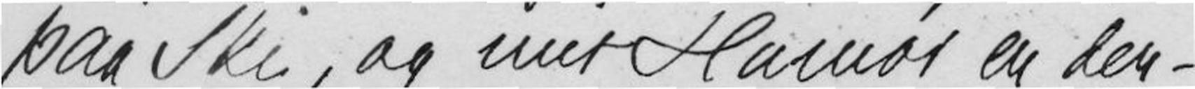paa Ski, og mit Humør er der-
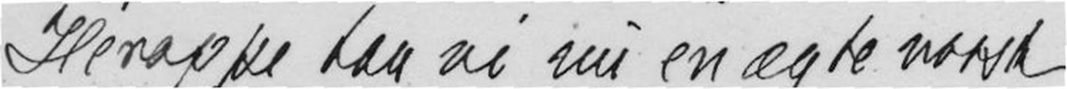Heroppe har vi nu en ægte norsk
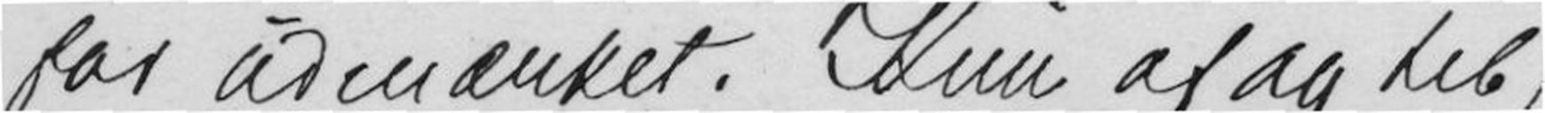for Udmærket. Kun af og til,
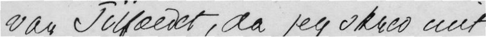var Tilfældet, da jeg skrev mit
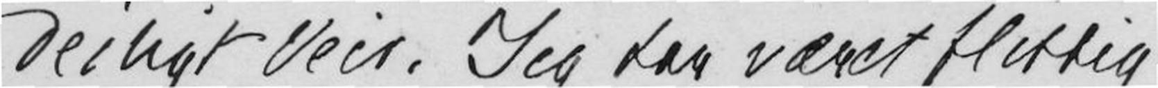Deiligt Veir. Jeg har været flittig
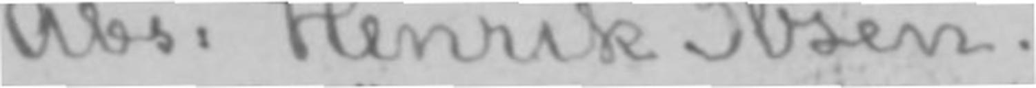Abs: Henrik Ibsen.
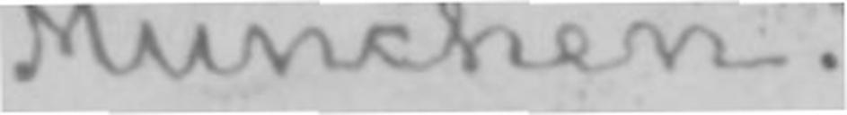München.
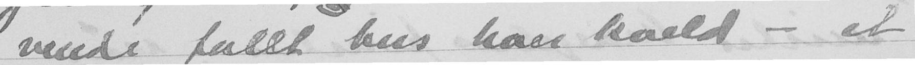vende fullt hus hver kveld - et
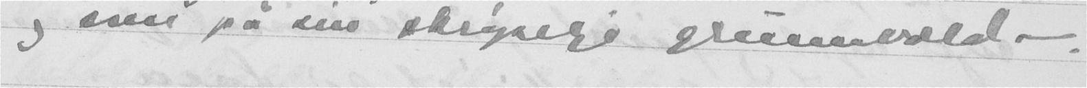og sover på sin skrøpelige grunnvold -
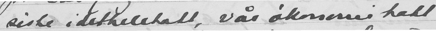siste idetheletatt, vår økonomi tatt
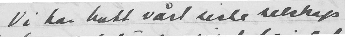Vi har hatt vårt siste selskap
